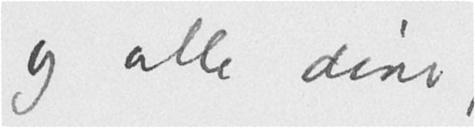og alle dine,
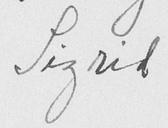Sigrid
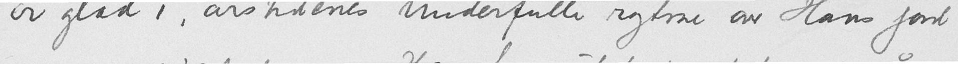er glad i, årstidenes underfulle rytme av Hans jord,
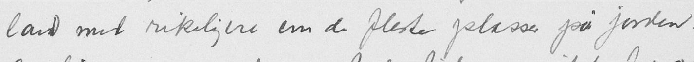land med rikeligere en de fleste plasser på jorden.
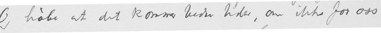Og håber at det kommer bedre tider, om ikke for oss
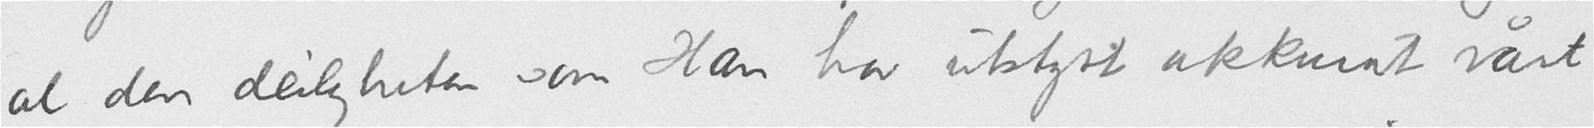al den deiligheten som Han har utstyrt akkurat vårt
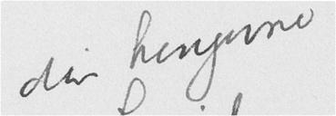din hengivne
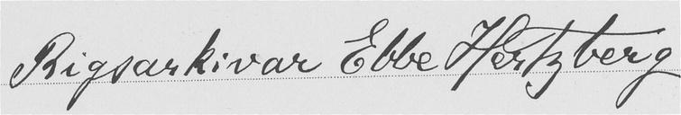Rigsarkivar Ebbe Hertzberg
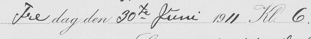Fredag den 30te Juni 1911 Kl. 6.
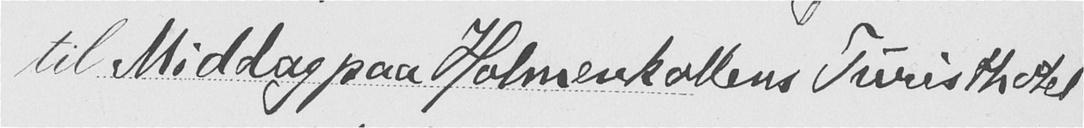til Middag paa Holmenkollens Turisthotel
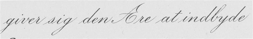giver sig den Ære at indbyde
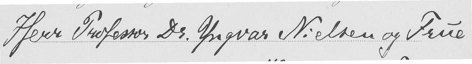Herr Professor Dr. Yngvar Nielsen og Frue
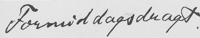Formiddagsdragt.
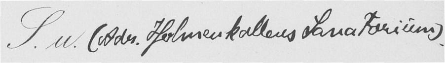S. u. (Adr. Holmenkollens Sanatorium).
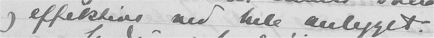og effektive ved hele anlegget.
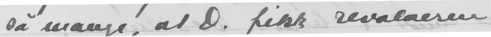så mange, at D. fikk revolveren
